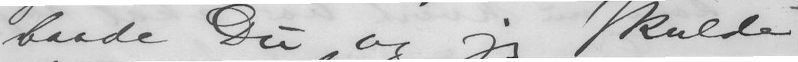baade Du og jeg skulde
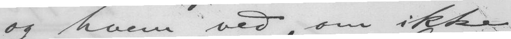og hvem ved, om ikke
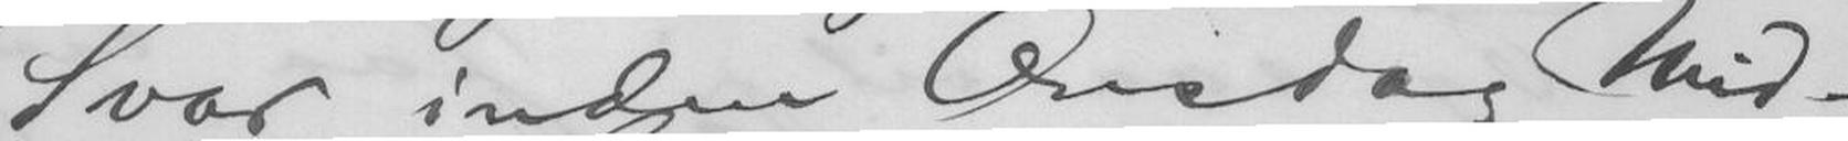Svar inden Onsdag Mid-
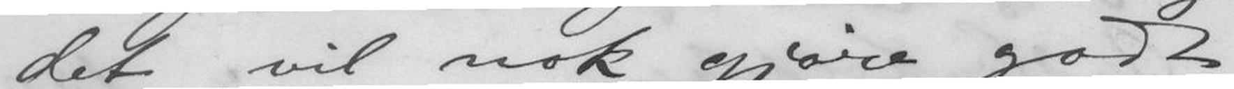det vil nok gjøre godt
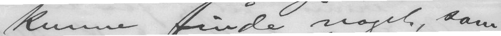kunne finde noget, som
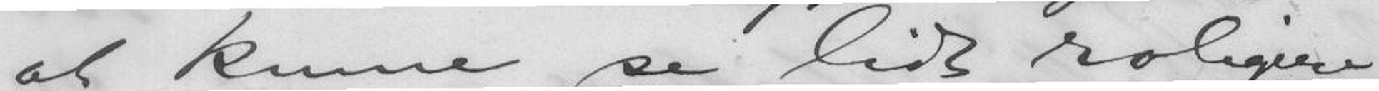at kunne se lidt roligere
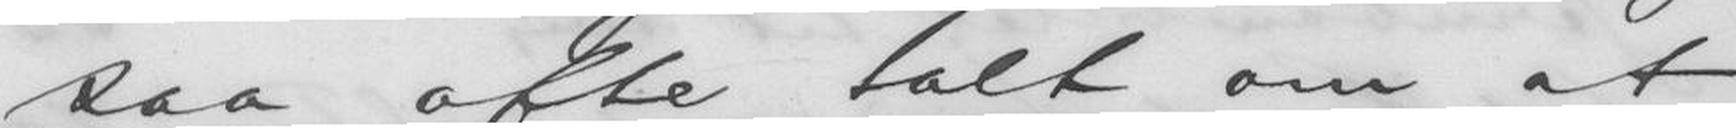saa ofte talt om at
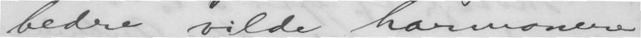bedre vilde harmonere
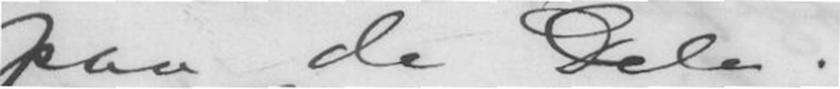paa de Dele.
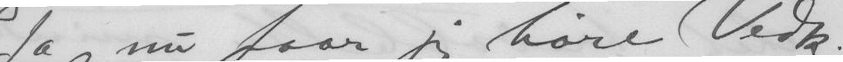Ja nu faar jeg høre Vedk.
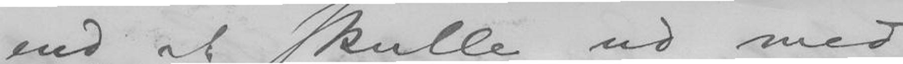end at skulle ud med
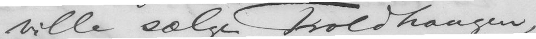ville sælge Troldhaugen,
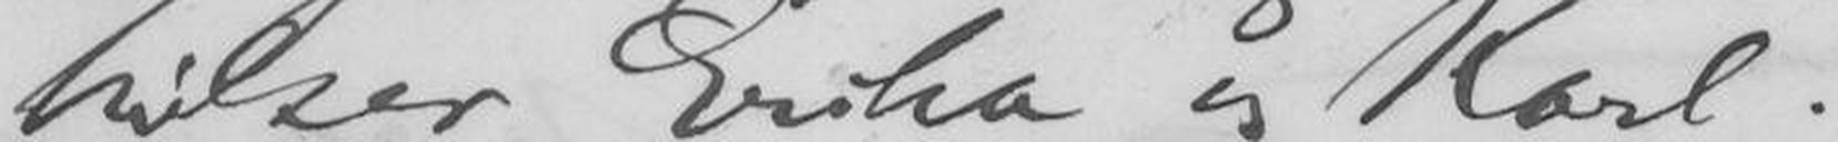hilser Erika og Karl.
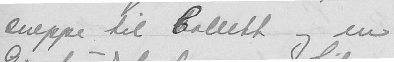sneppe til Collett og en
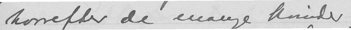hvorefter de mange kvinder,
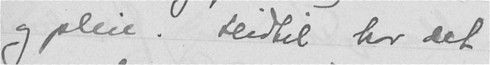og pleie. Hidtil har det
